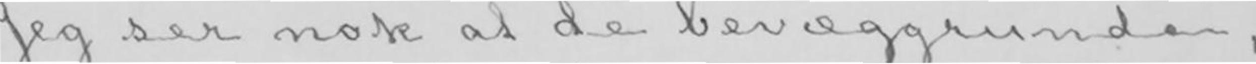Jeg ser nok at de bevæggrunde,
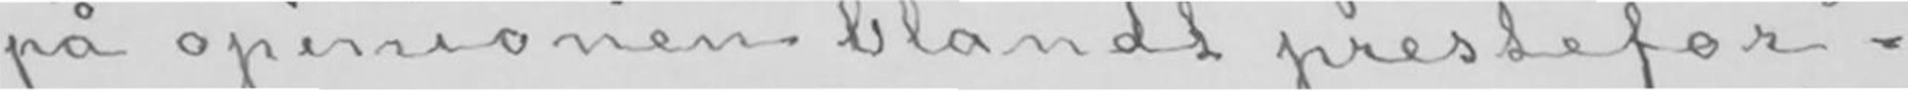på opinionen blandt prestefor-
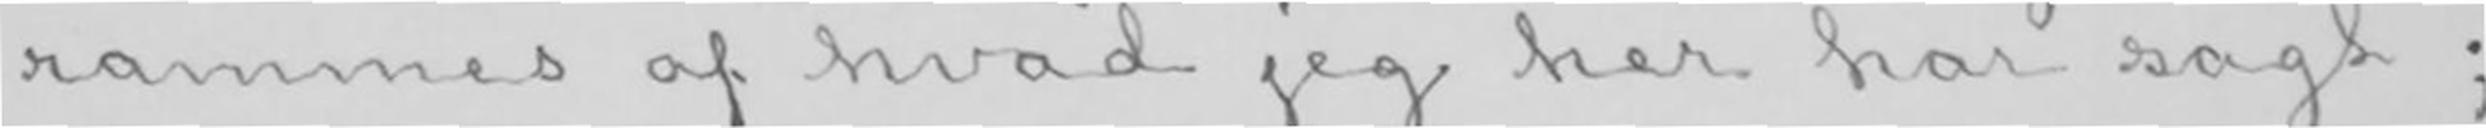rammes af hvad jeg her har sagt;
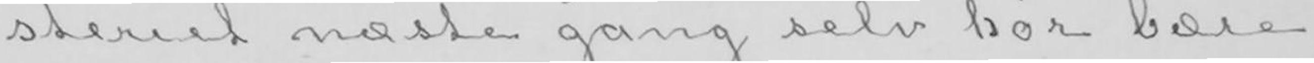steriet næste gang selv bør bære
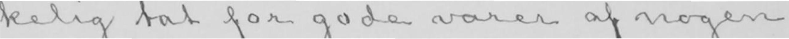kelig tat for gode varer af nogen
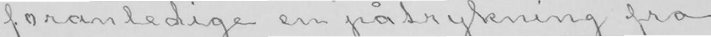foranledige en påtrykning fra
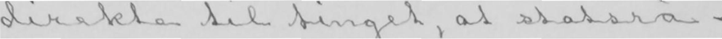direkte til tinget, at statsrå-
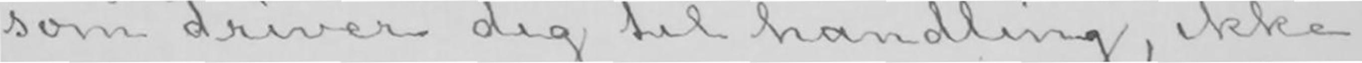som driver dig til handling, ikke
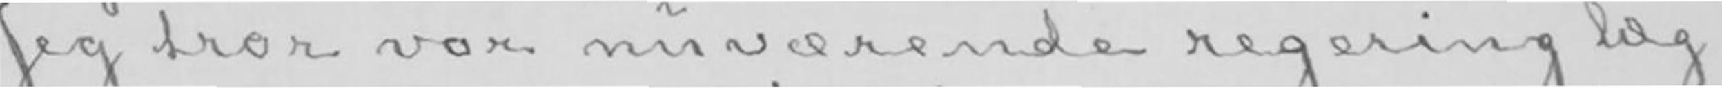Jeg tror vor nuværende regering læg-
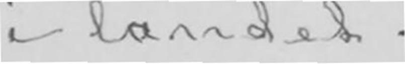i landet.
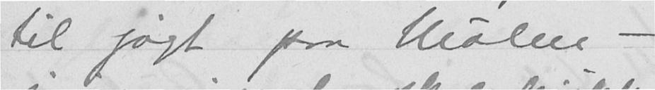til jagt paa Mølen -
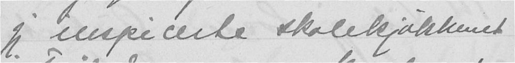jeg inspicerte skolekjøkkenet
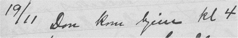19/11 Don kom hjem kl 4
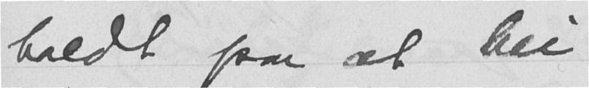holdt paa at bli
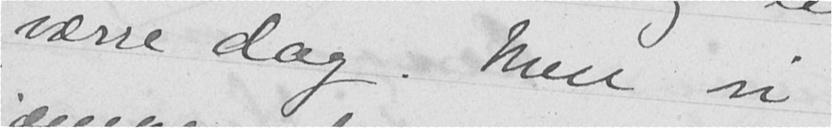værre dag. Men vi
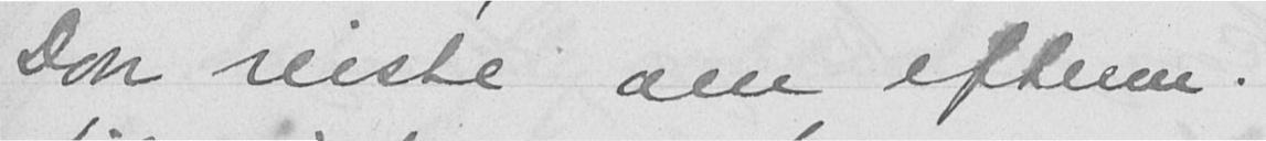Don reiste om efterm.
